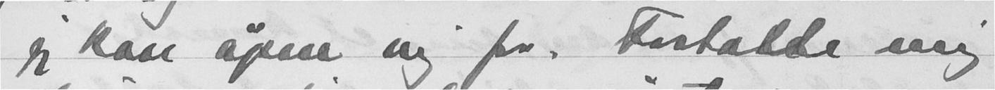jeg kan åpne mig for. Fortalte mig
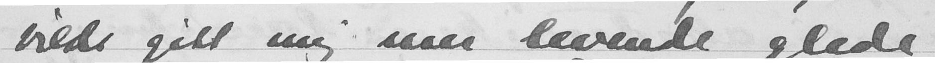vilde gitt mig mer levende glede
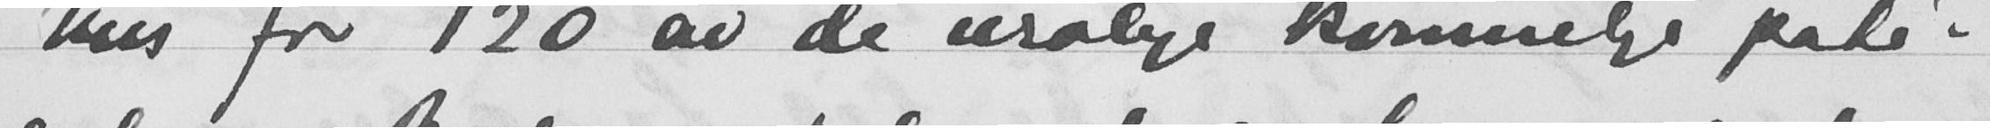hus for 120 av de urolige kvinnelige pati-
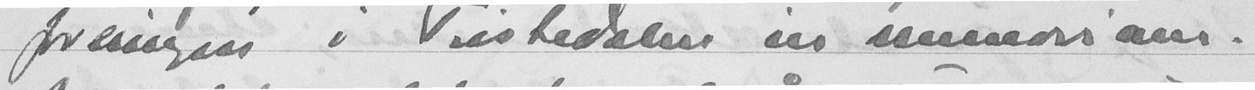foreningen i Tristedalen in memoriam.
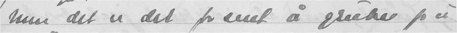Men det er det forsent å gruble på
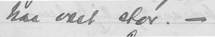har vært stor. -
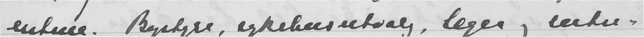entene. Bystyre, sykehusutsalg, leger og lutre-
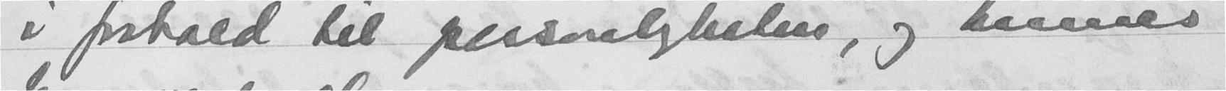i forhold til personligheten, og krisen
